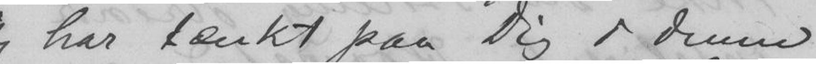jeg har tænkt paa Dig i denne
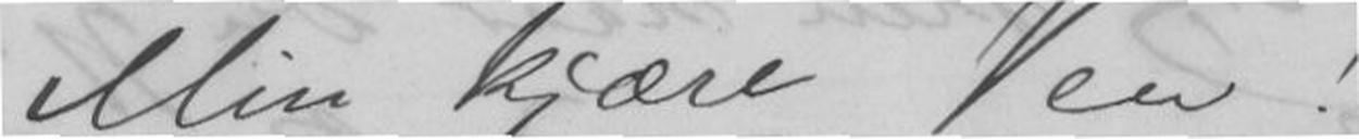Min kjære Ven!
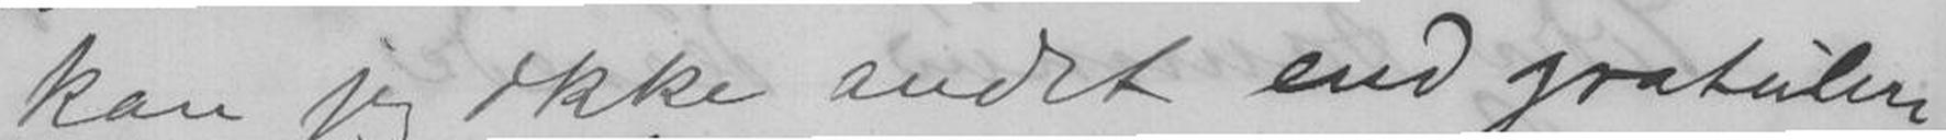kan jeg ikke andet end gratulere
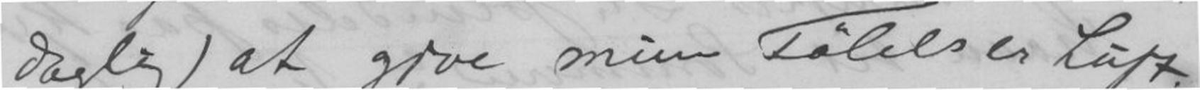daglig) at give mine Følelser Luft.
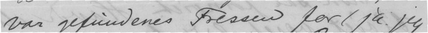var gefundenes Fressen for, ja jeg
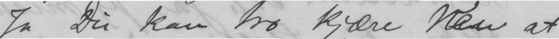Ja Du kan tro kjære Ven at
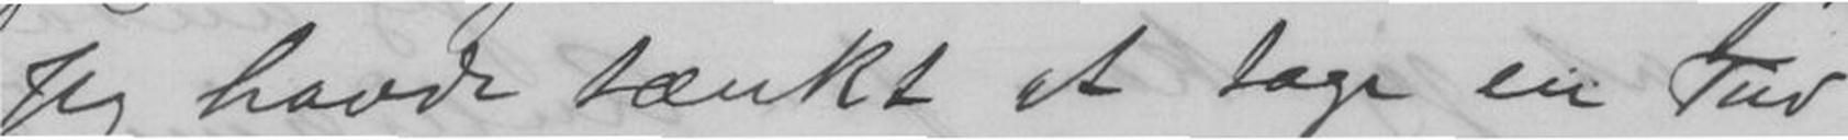Jeg havde tænkt at tage en Tur
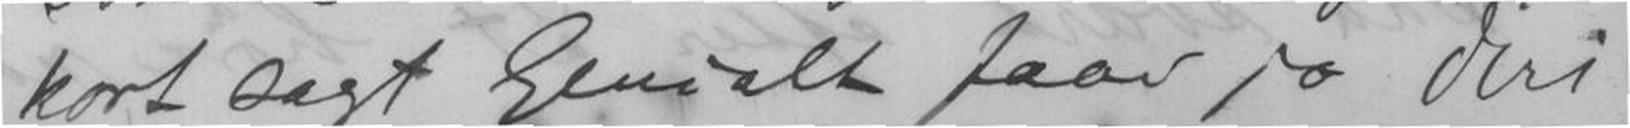kort sagt Genialt faar jo Deri
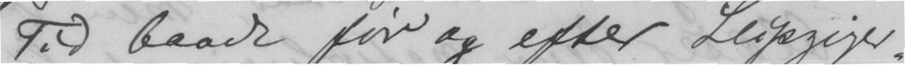Tid baade før og efter Leipziger-
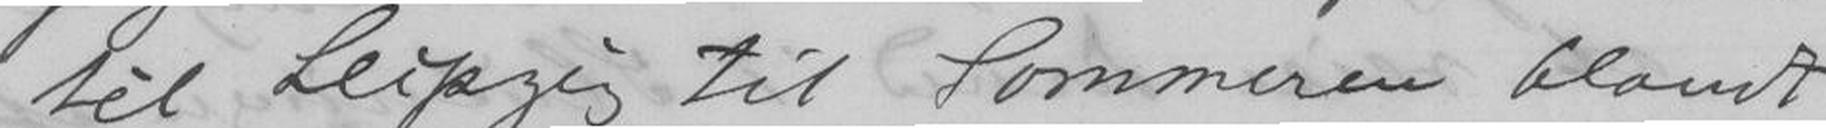til Leipzig til Sommeren blandt
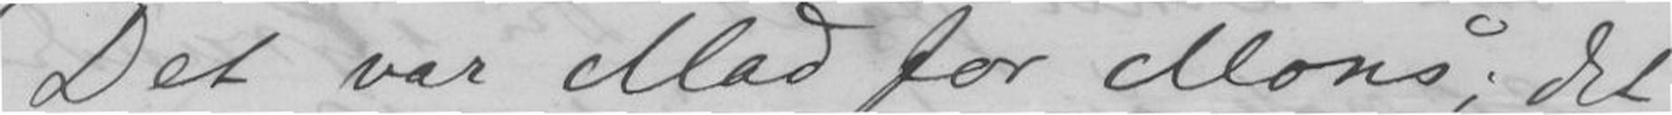Det var Mad for Mons; det
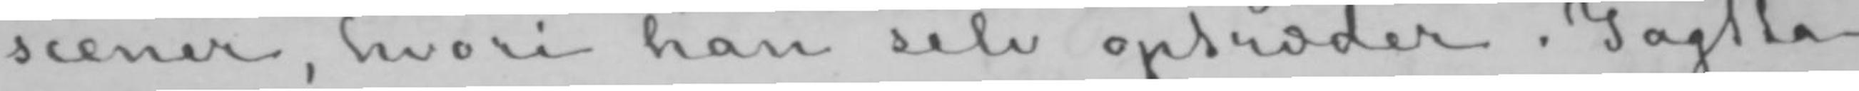scener, hvori han selv optræder. Iagtta-
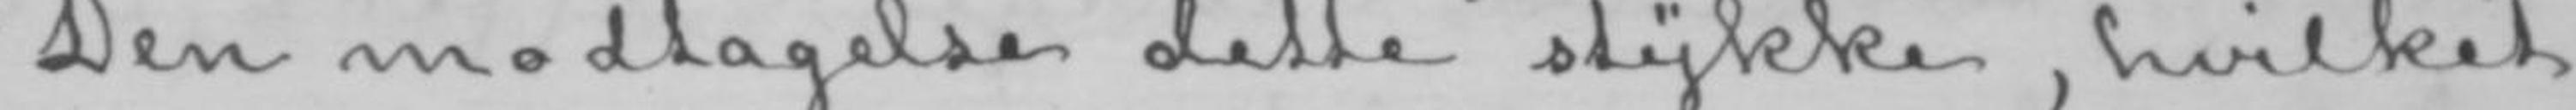Den modtagelse dette stykke, hvilket
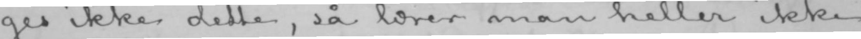ges ikke dette, så lærer man heller ikke
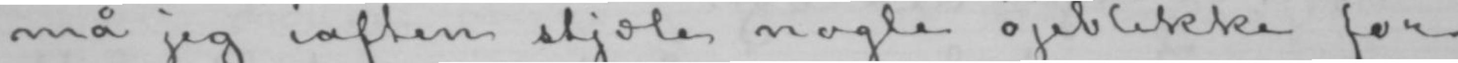må jeg iaften stjæle nogle øjeblikke for
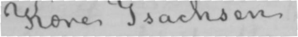Kære Isachsen!
